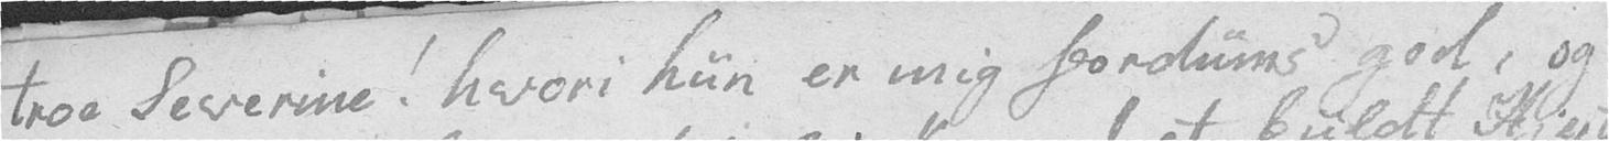troe Severine! hvori hun er mig fordums god, og
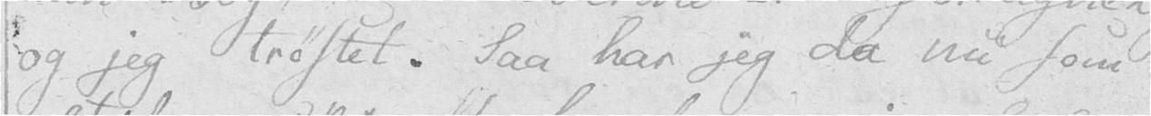og jeg trøstet. Saa har jeg da nu som
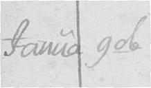Janua 9de
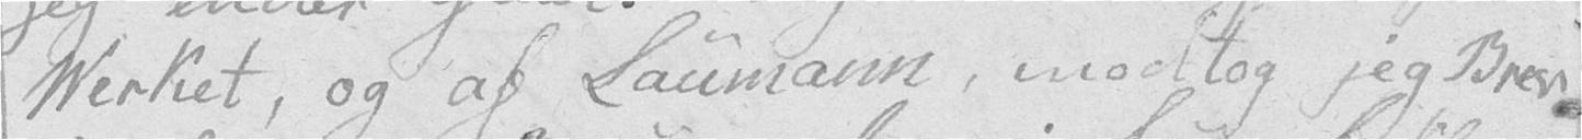Verket, og af Laumann, modtog jeg Brev
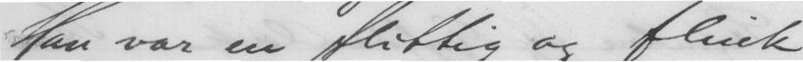Han var en flittig og flink
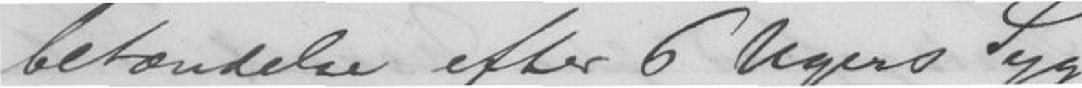betændelse efter 6 Ugers Syg-
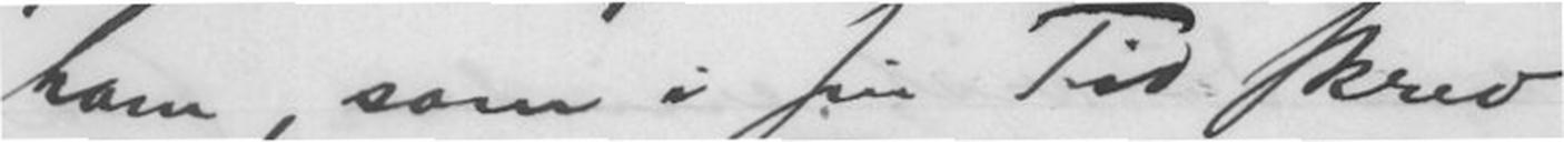ham, som i sin Tid skrev
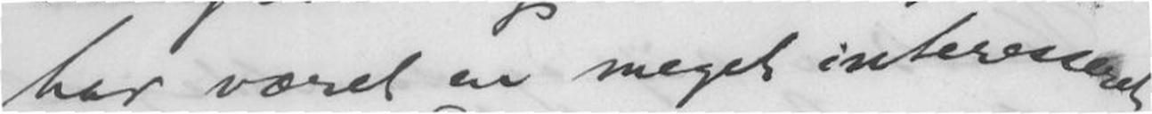har været en meget interesseret
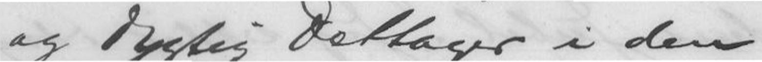og dygtig Deltager i den
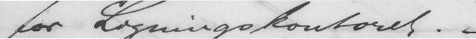for Ligningskontoret. -
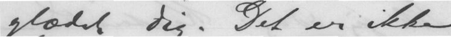glædet dig. Det er ikke
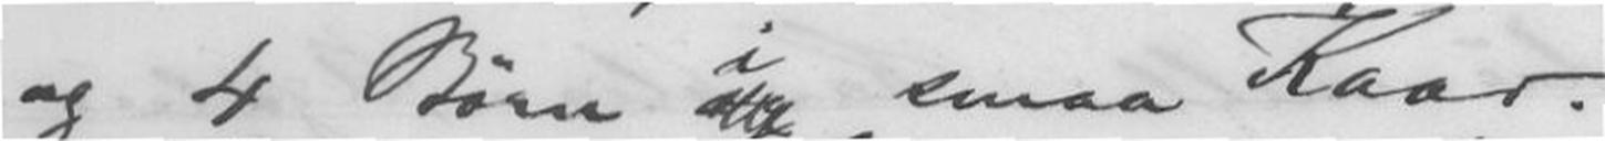og 4 Børn og i smaa Kaar.
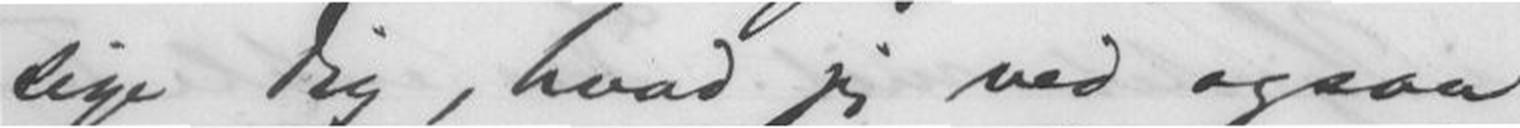sige Dig, hvad jeg ved ogsaa
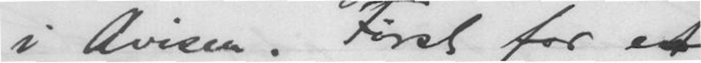i Avisen. Først for et
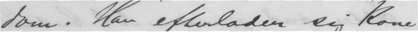dom. Han efterlader sig Kone
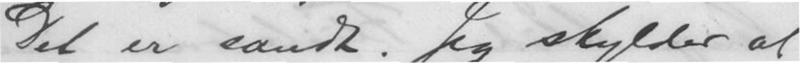Det er sandt. Jeg skylder at
